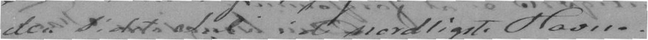den sidste Juli i de nordligste Havne.
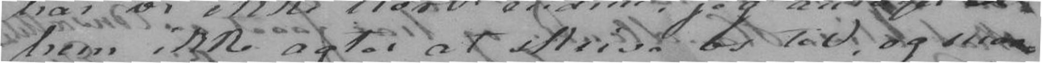hun ikke agter at skrive os til, og maa-
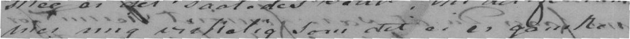mer mig virkelig som det ei er ganske
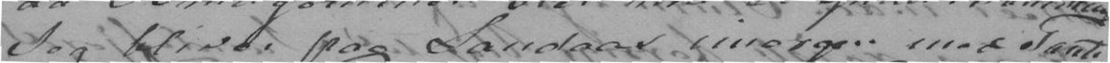Jeg bliver paa Landaas imorgen med Tante
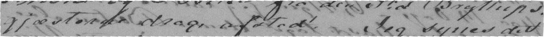gjæsterne drager afsted. Jeg synes det
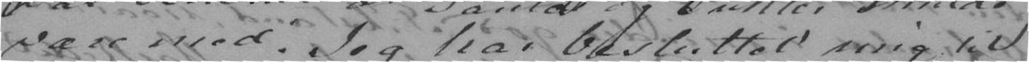være med. Jeg har besluttet mig til
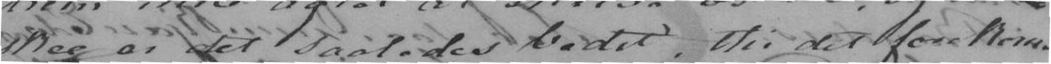skee er det saaledes bedst, thi det forekom-
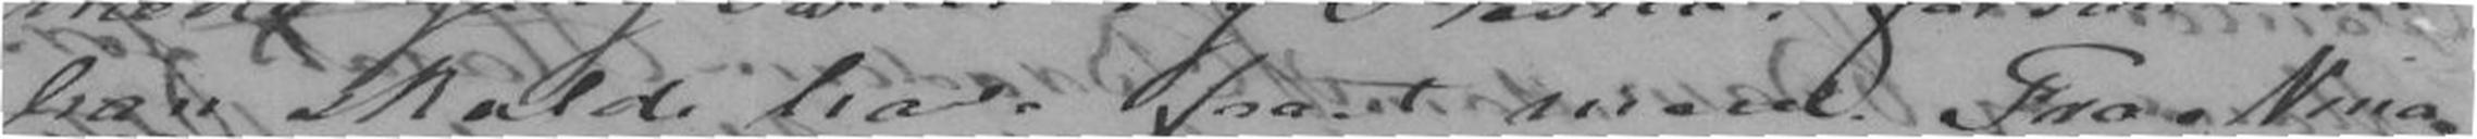han skulde have faaet mere. Fra Nina
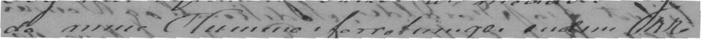da mine Hummer forretninger endnu ikke
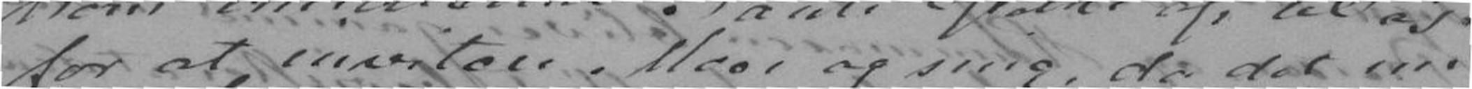for at invitere Moer og mig, da det nu
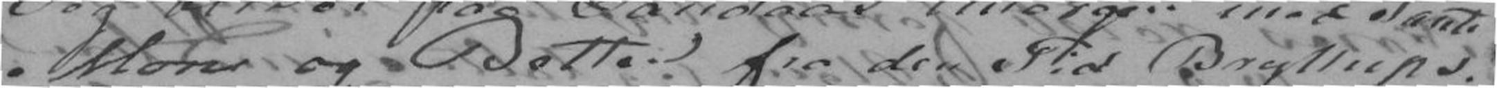Mone og Betten fra den Tid bryllups-
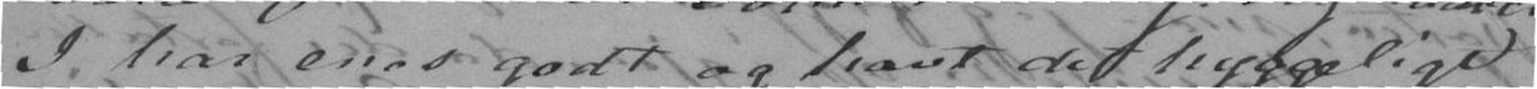I har enes godt og havt det hyggeligt
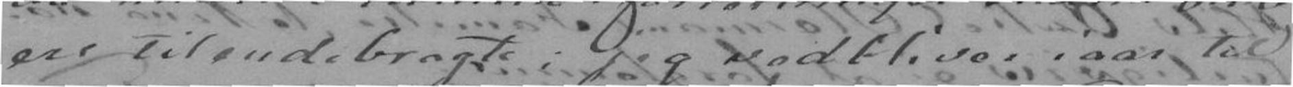ere tilendebragt; jeg vedbliver iaar til
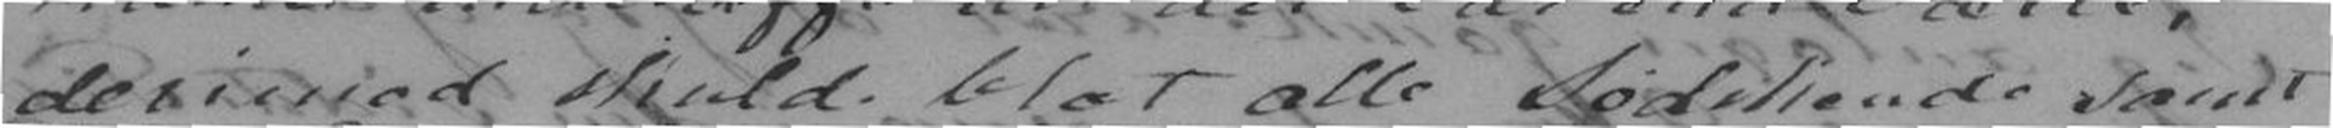derimod skulde blot alle Sødskende samt
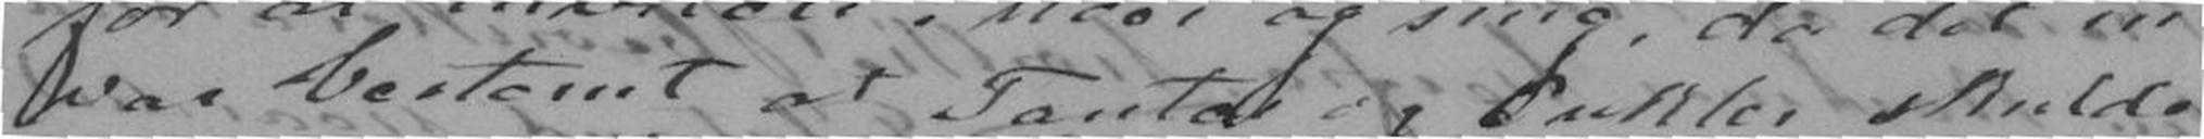var bestemt at Tanter og Onkler skulde
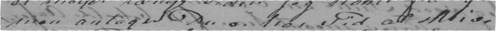men antager Du ei har Tid at skrive
